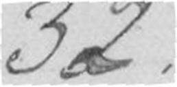32.
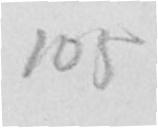105
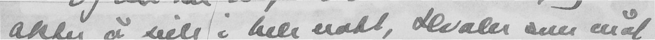akter å seile i hele natt, Hvaler som mål.
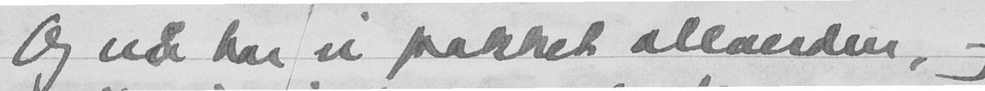Og nu har vi pakket allverden, og
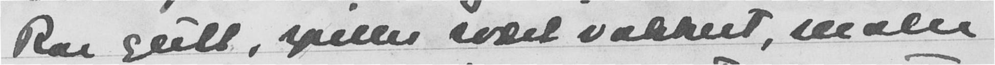Rar gutt, spiller svært vakkert, maler
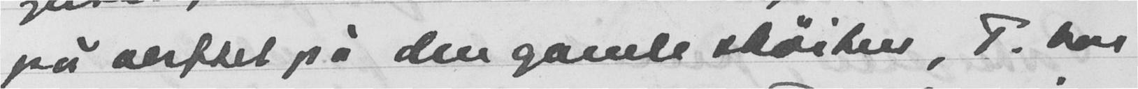på verftet på den gamle skøiken, F. har
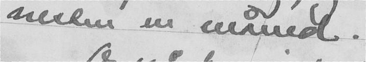nesten en måned.
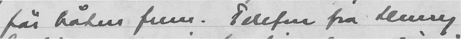får båten frem. Telefon fra Henry
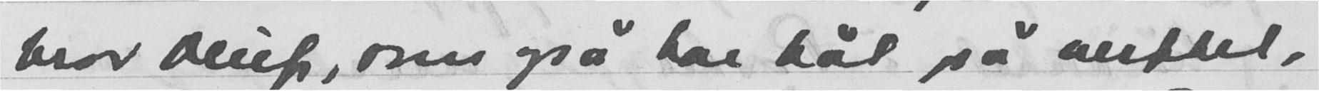bror Oluf, som også har båt på verftet,
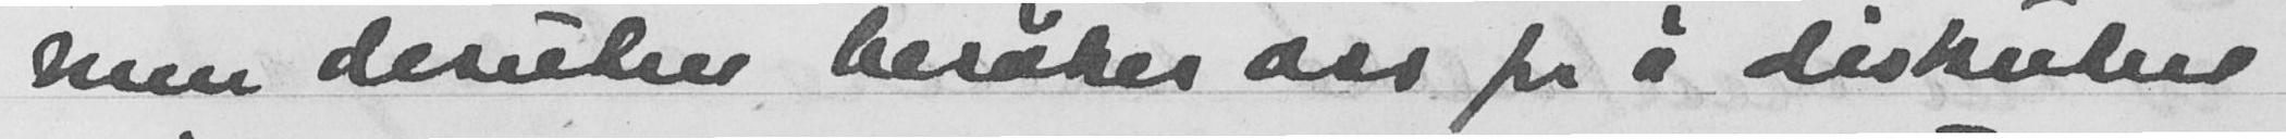men desuten besøker oss for å diskutere
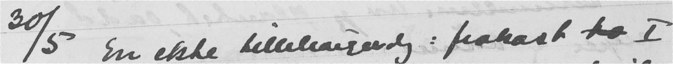30/5 En ekte tillehangerdag: frokost  I
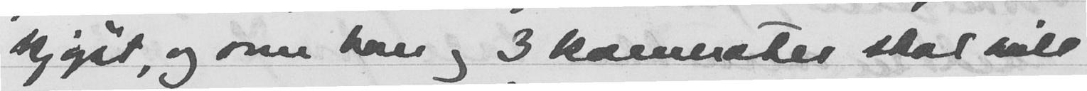kjøpt, og som han og 3 kamerater skal seile
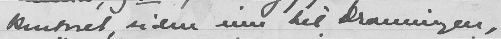kontoret, siden inn til Dronningen,
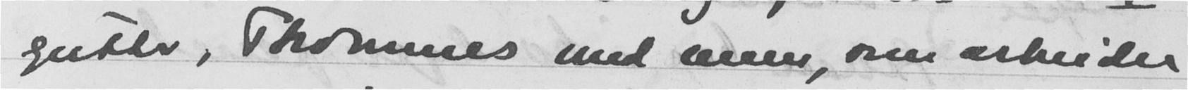gutter, Thommes med menn, som arbeider
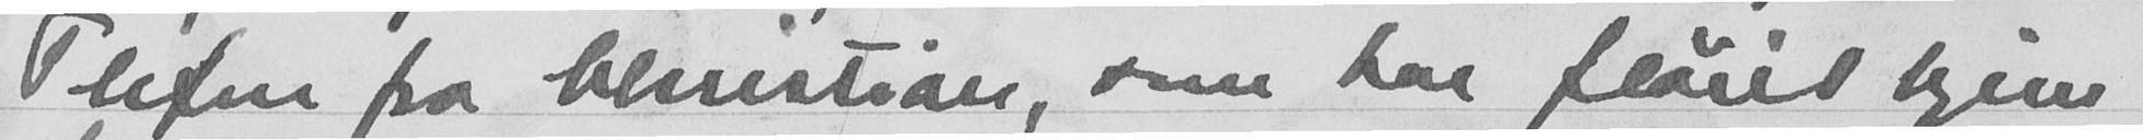Telefon fra Christian, som har fløiet hjem
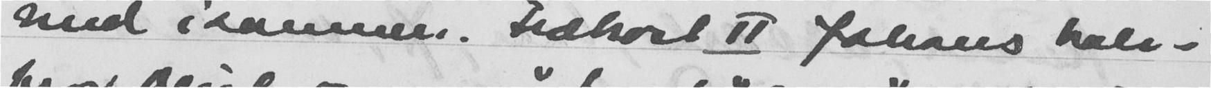med isammen. Frokost II Johans halv-
